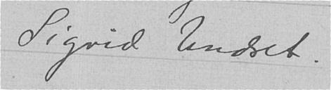Sigrid Undset.
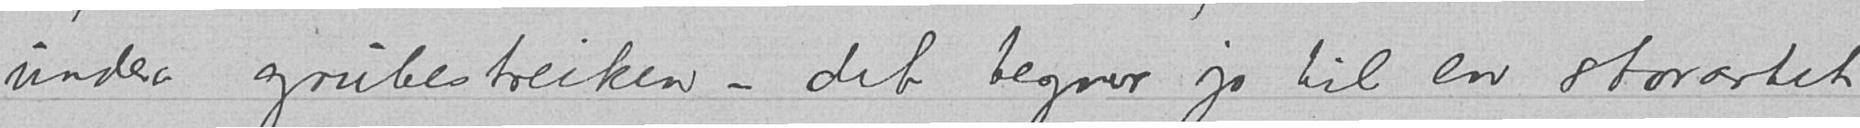under grubestreiken - det tegner jo til en storartet
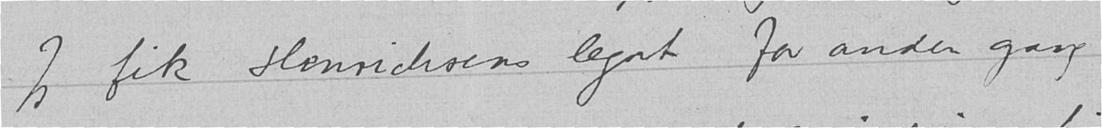- Jeg fik Henrichsens legat for anden gang
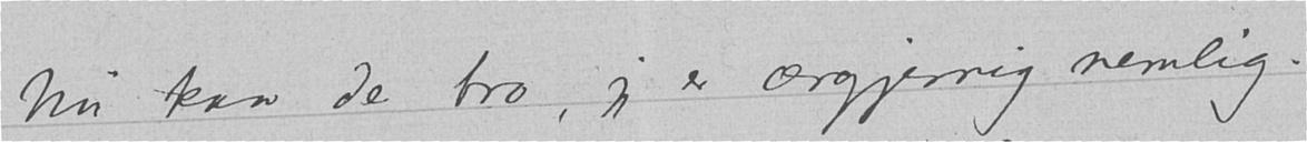Nu kan de tro, jeg er ærgjernig nemlig.
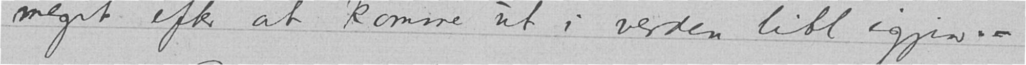meget efter at komme ut i verden litt igjen. –
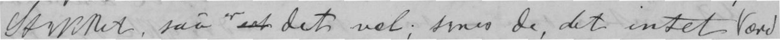Stykket, saa er det vel; synes De, det intet Værd
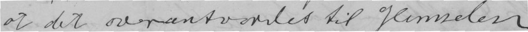at det overantvordes til Himmelen.
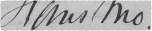Hans Mo.
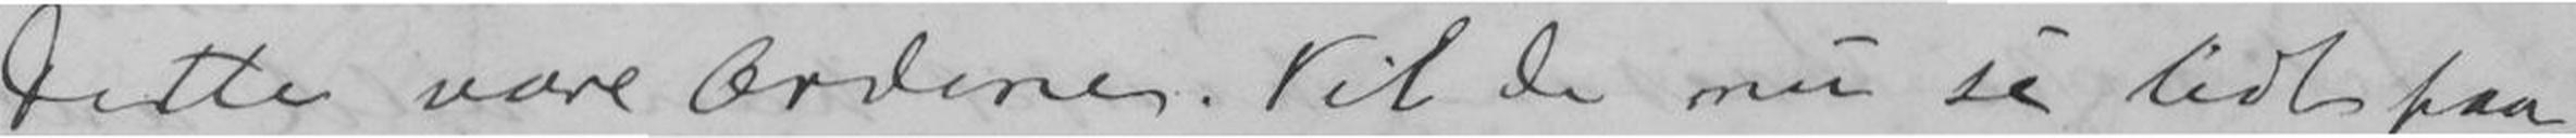Dette være Ordene. Vil De nu se lidt paa
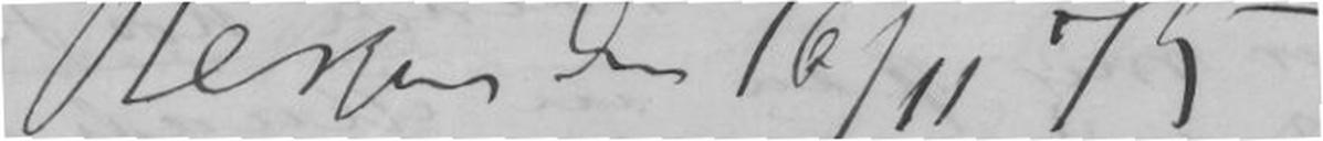Bergen den 16/11 75
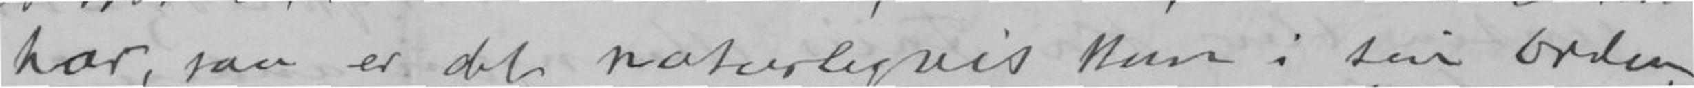har, saa er det naturligvis kun i sin orden,
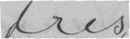Deres
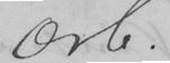Ærb.
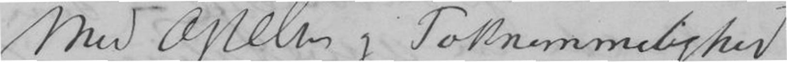Med Hilsen og Taknemmelighed
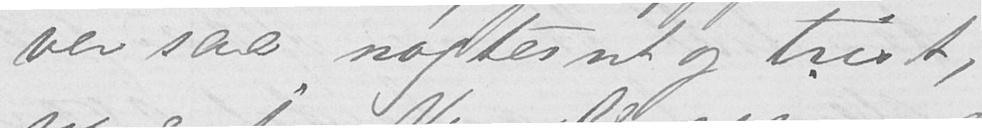ver saa nøgternt og trist,
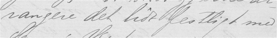rangere det lidt festligt med
